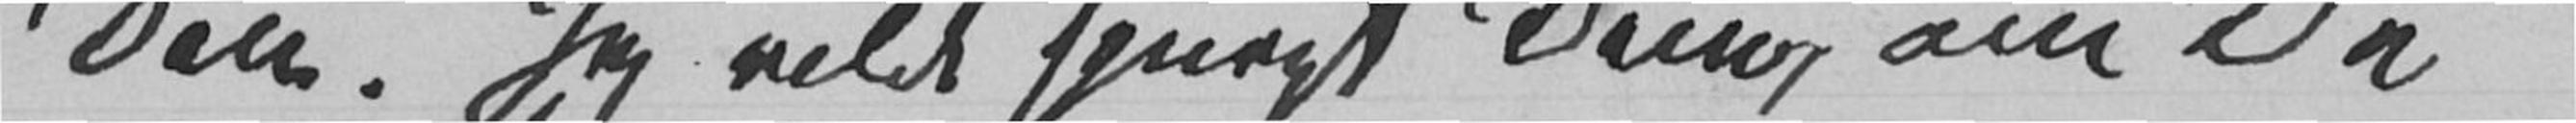Dem. Jeg vilde spurgt Dem, om De
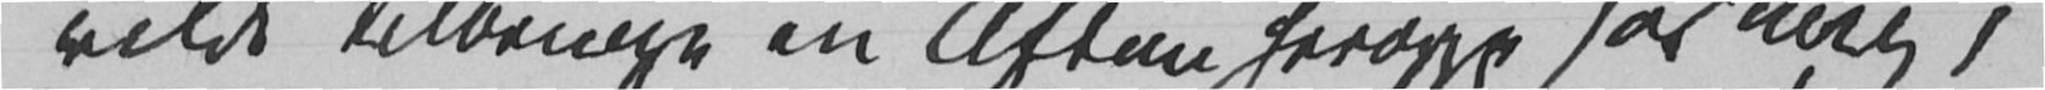vilde tilbringe en Aften heroppe hos mig,
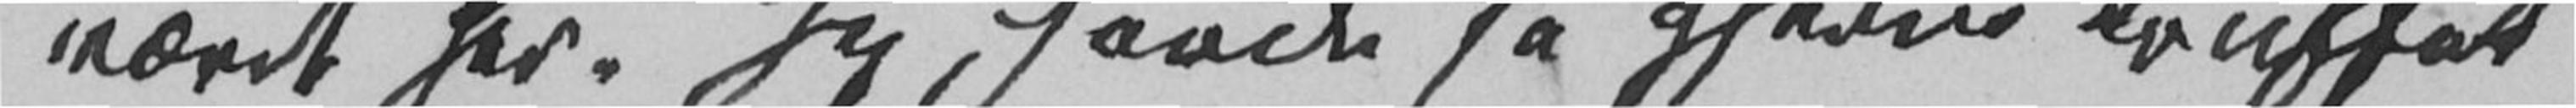været her. Jeg havde så gjerne truffet
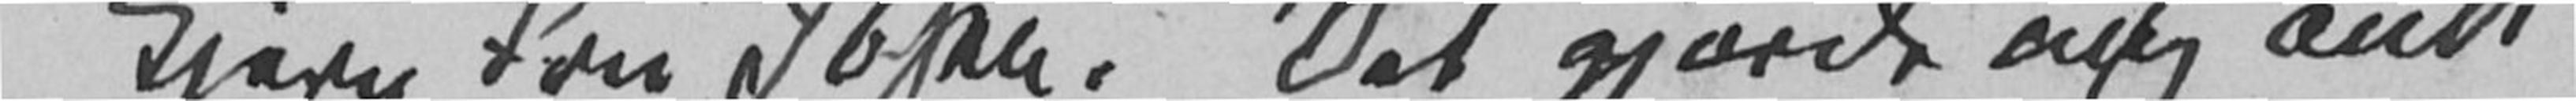Kjere Fru Ibsen! Det gjorde mig ondt
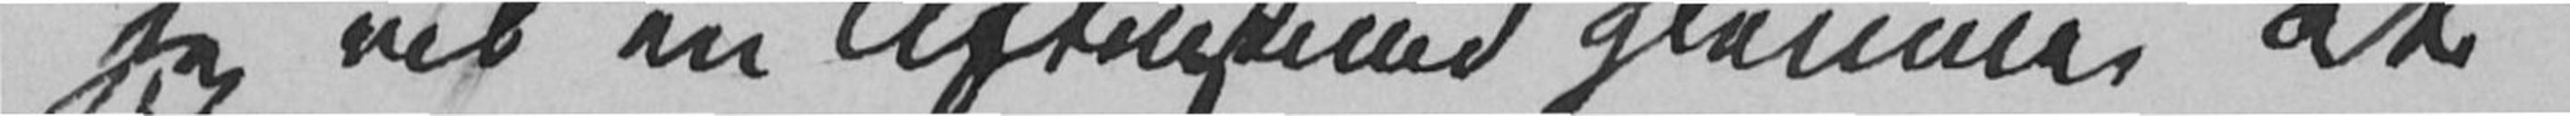jeg vil en Aftenstund glemme at
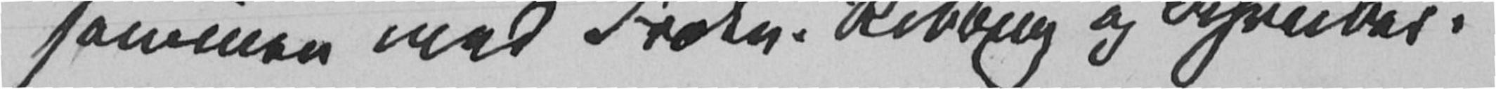sammen med Frøken Ribbing og Schreiber.
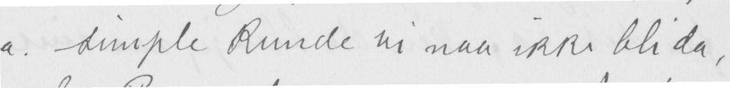simple kunde vi naa ikke bli da,
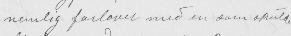nemlig forlovet med en som skulde
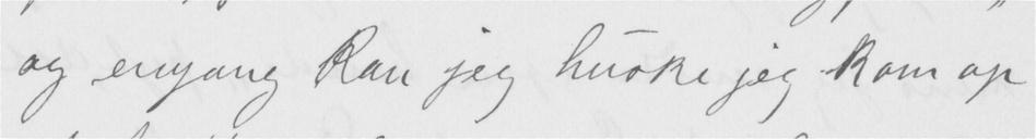og engang kan jeg huske jeg kom op
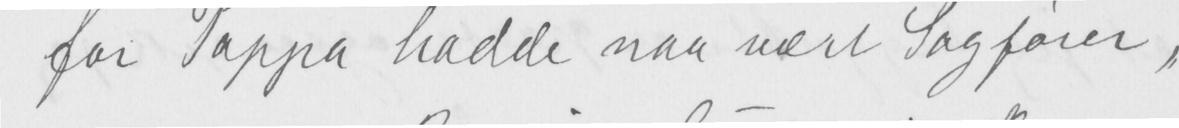for Pappa hadde naa vært Sagfører,
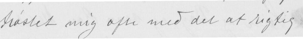trøstet mig ofte med det at rigtig
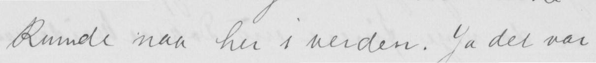kunde naa her i verden. Ja det var
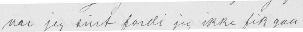var jeg sint fordi jeg ikke fik gaa
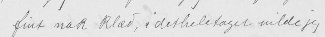fint nok klæd, i detheletaget vilde jeg
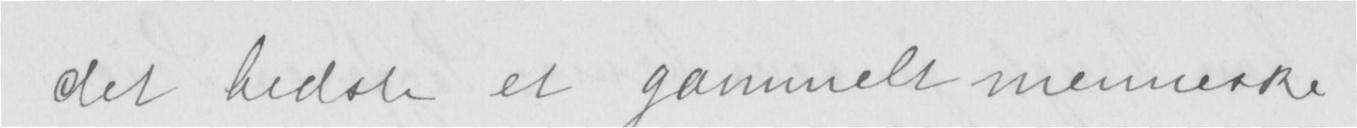det bedste et gammelt menneske
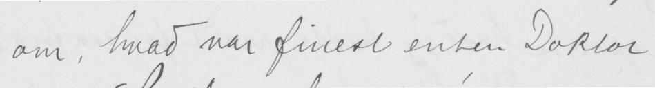om, hvad var finest enten Doktor
